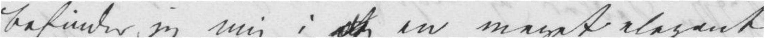befinder jeg mig i et</del> en meget elegant
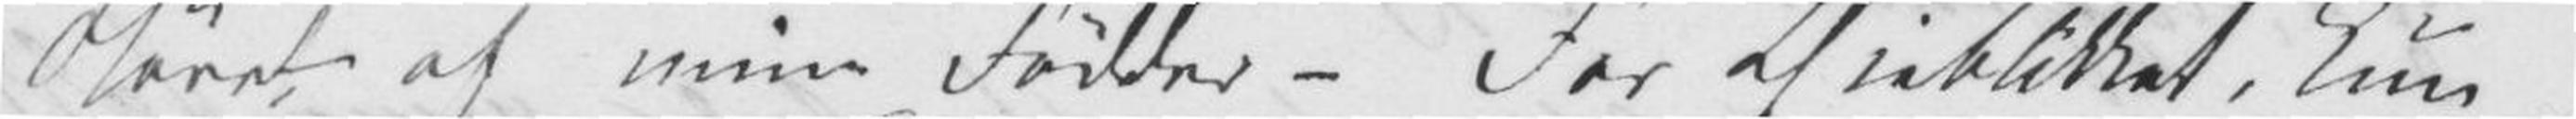Støvet af mine Fødder – For Øieblikket, kun
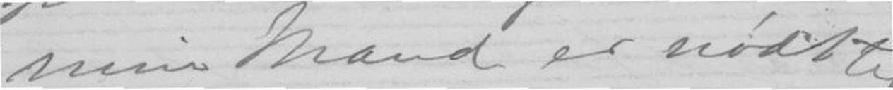min Mand er nødt til
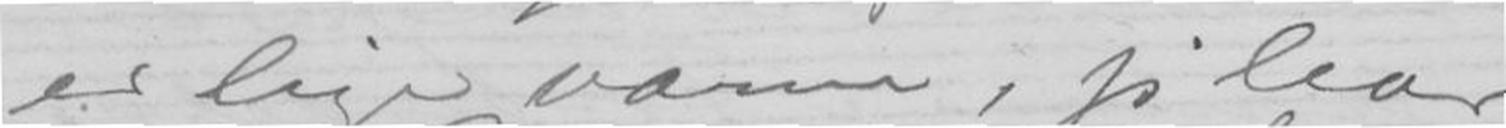er lige varm, jeg lever
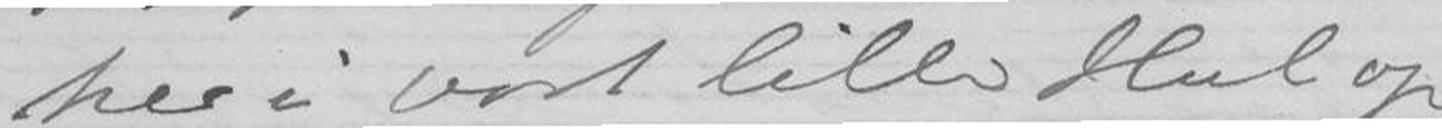her i vort lille Hul op-
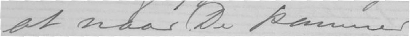at naar De kommer
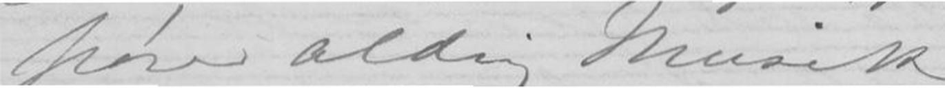høre aldrig Musik.
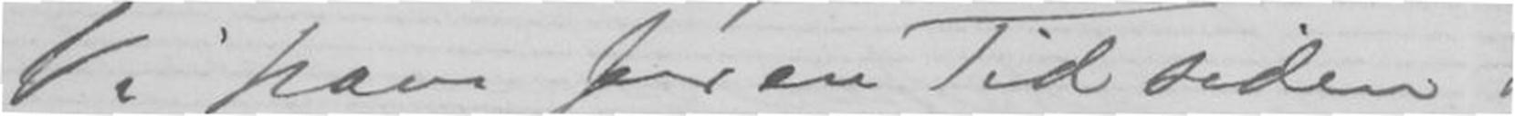Vi have for en Tid siden
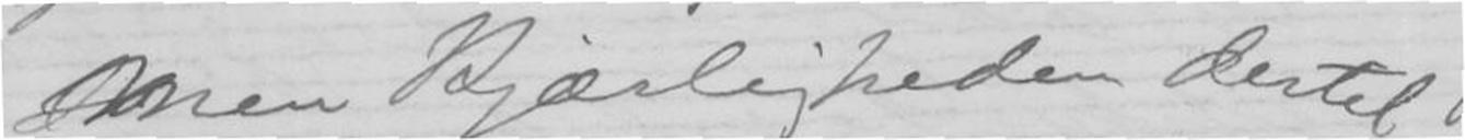Men Kjærligheden dertil
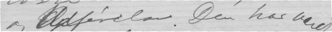og Udførelse. Den har været
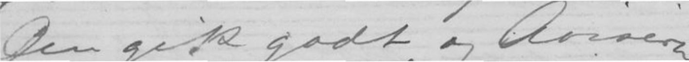Den gik godt og Aviserne
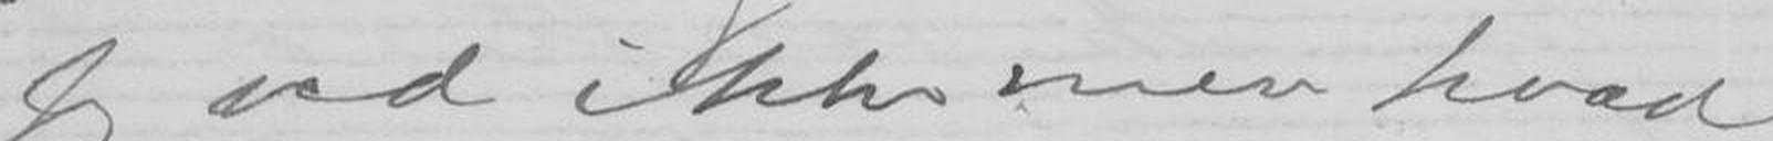Jeg ved ikke mere hvad
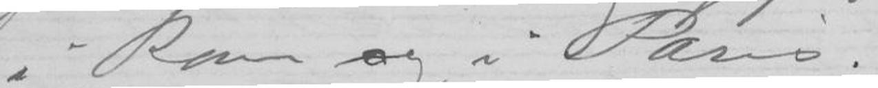i Rom og i Paris.
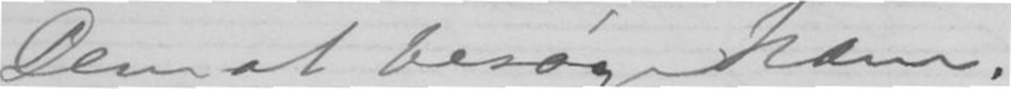Dem at besøge ham.
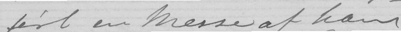ført en Messe af ham.
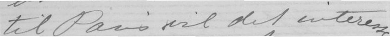til Paris vil det interessere
